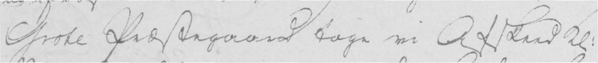Grote Præstegaard toge vi Afskeed Kl.
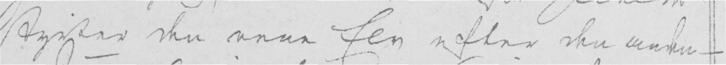styrter den eene Elv efter den anden -
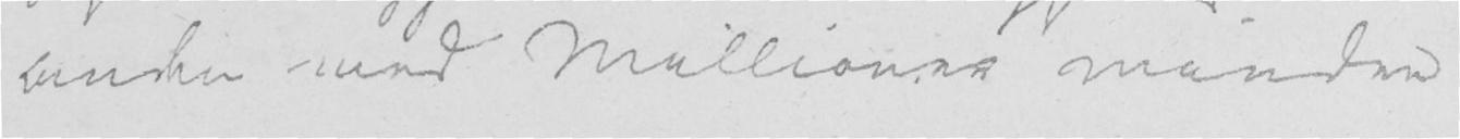anden med Millioner mindre
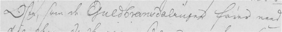Oste, som de Guldbransdalinger fører need
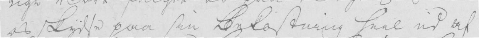os Skydse paa sin Bekostning heel ud af
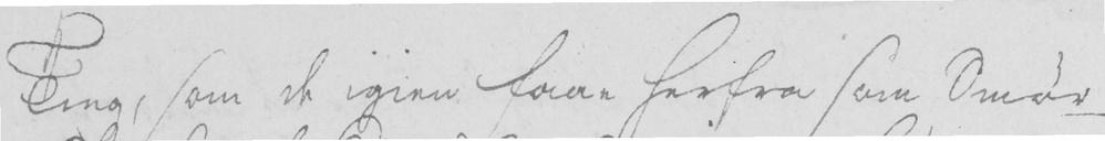Ting, som de igien faae herfra som, Smør
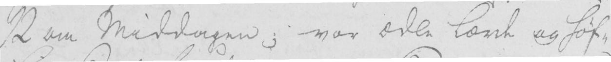12 om Middagen; vor ædle lærde og høf-
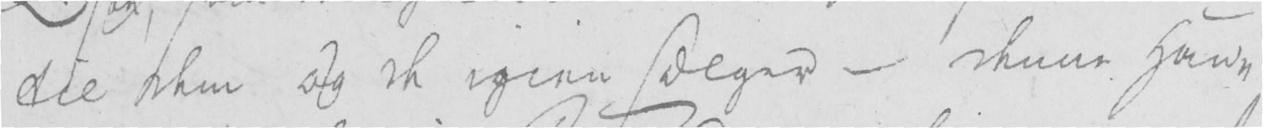til dem og de igien sælger - Denne Han-
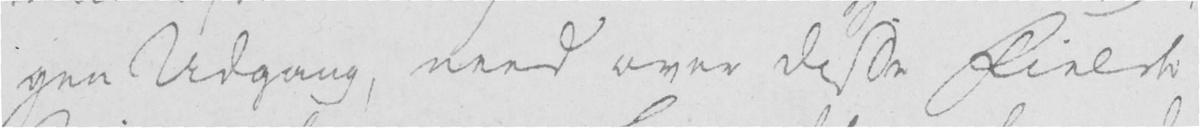gen Udgang, need over disse Fielde
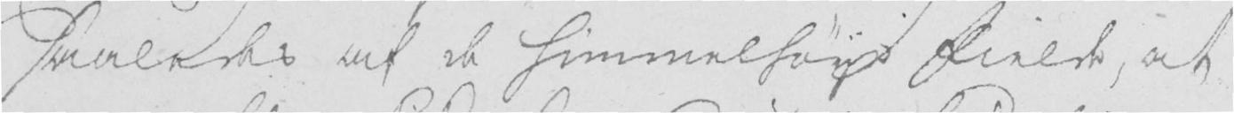saaledes af de himmelhøye Fielde, at
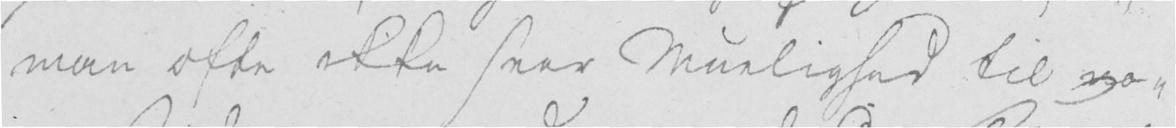man ofte ikke seer Muelighed til no-
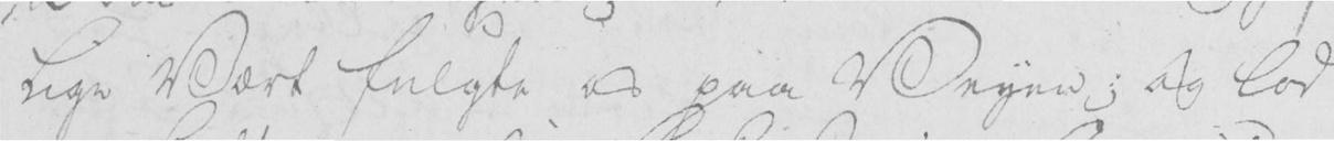lige Vært fulgte os paa Veyen; og lod
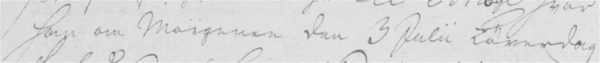han om Morgenen den 3 Julii Løverdag
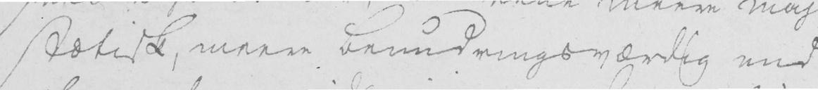stætisk, meere beundringsværdig end
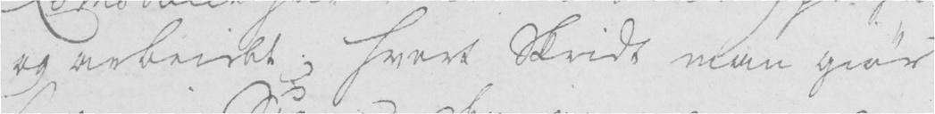og arbeidet; hvert Skridt man giør
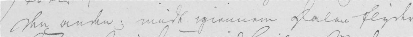den anden; midt igiennem Dalen flyder
